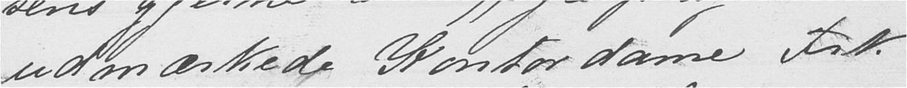udmærkede Kontordame Frk
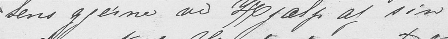tens gjerne ved Hjælp af sin
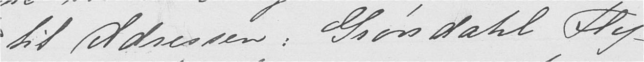til Adressen: Grøndahl Fly-
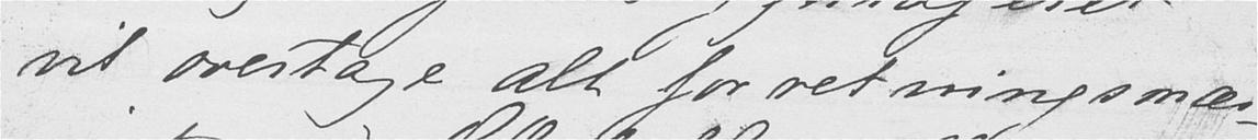vil overtage alt forretningsmæs-
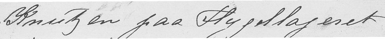Knutzen paa Flygellageret
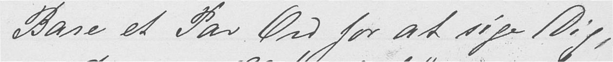Bare et Par Ord for at sige Dig,
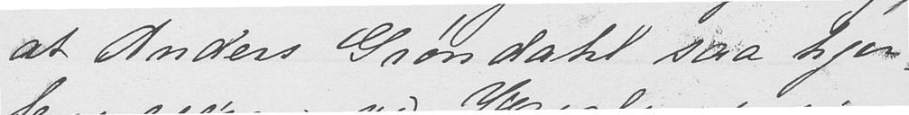at Anders Grøndahl saa hjer-
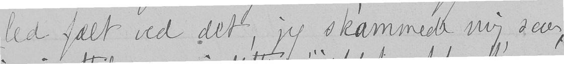led fælt ved det, jeg skammede mig saa,
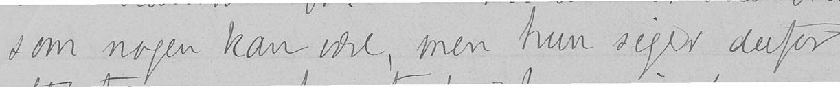som nogen kan være, men hun siger derfor
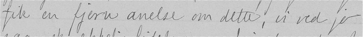fik en fjern anelse om dette, vi ved jo
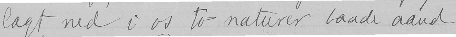lagt ned i os to naturer baade aand
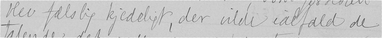blev fælslig kjedeligt, der vilde ialfald de
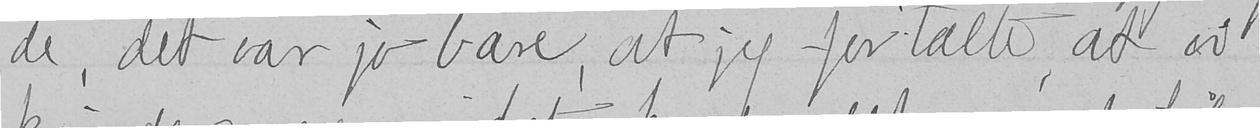de, det var jo bare, at jeg fortalte, at vi
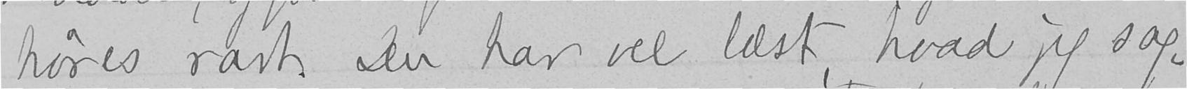høres rart. Du har vel læst, hvad jeg sag-
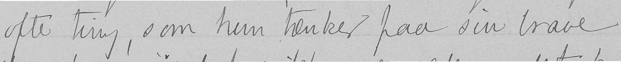ofte ting, som hun tænker paa sin brave
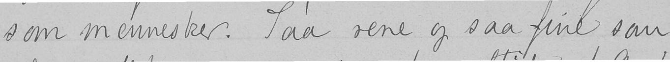som mennesker. Saa rene og saa fine som
